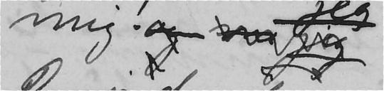mig! og nu jeg
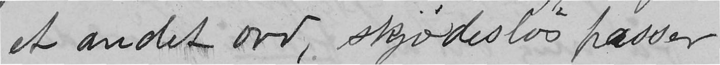et andet ord, skødesløs passer
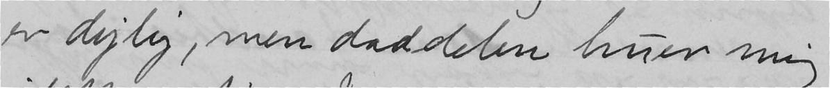er dejlig, men daddelen huer mig
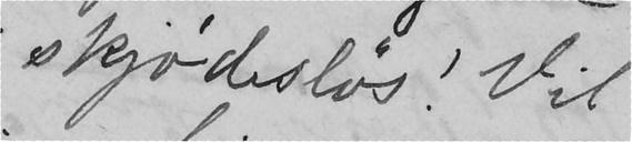skjødesløs! Vil
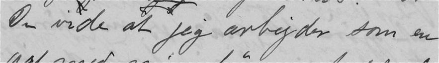Du vide at jeg arbejder som en
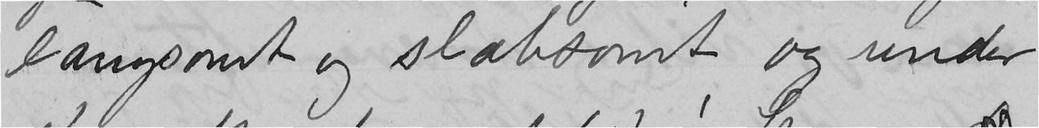langsomt og slæbsomt, og under
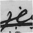jeg
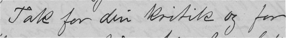Tak for din kritik og for
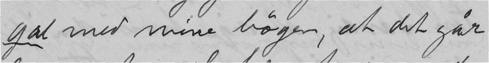gal med mine bøger, at det går
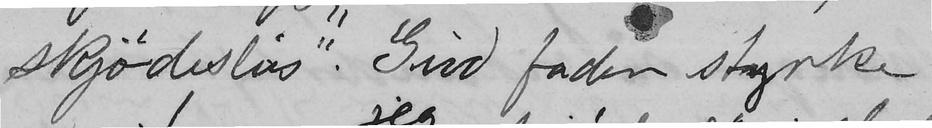skjødesløs". Gud fader styrke
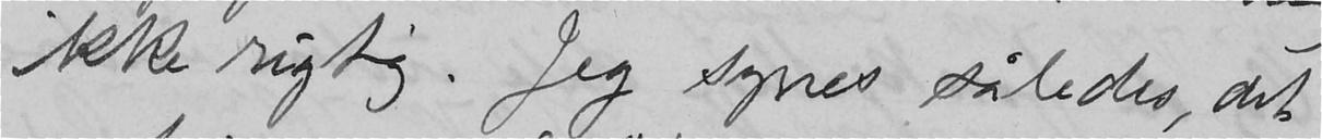ikke rigtig. Jeg synes således, det
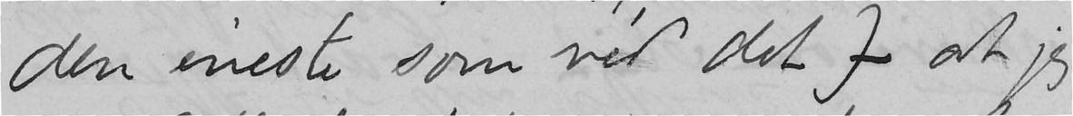den éneste som véd det) - at jeg
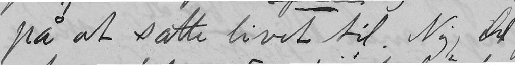på at sætte livet til. Nej, Du
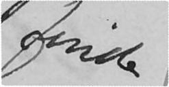finde
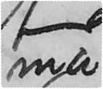må
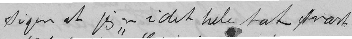siger at jeg "er idet hele tat svært
